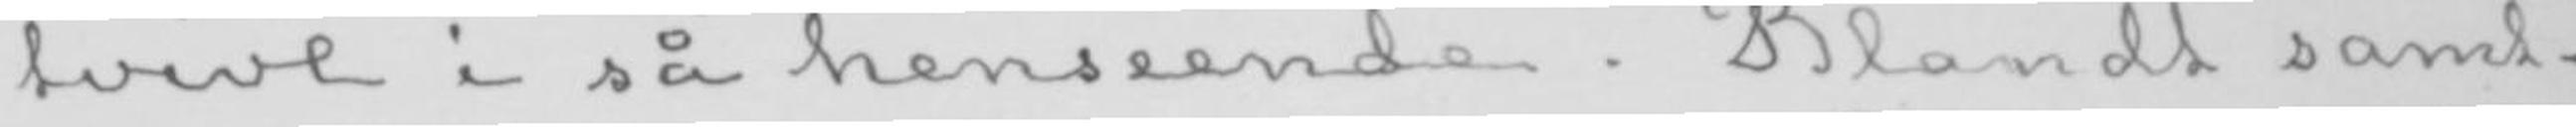tvivl i så henseende. Blandt samt-
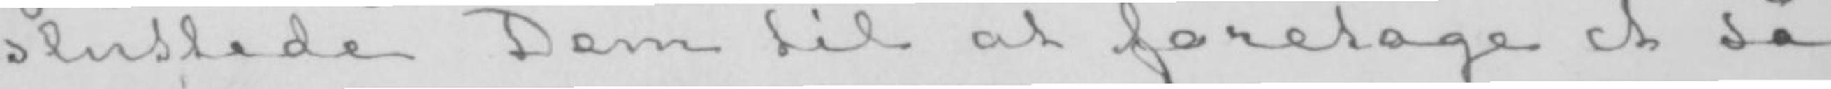sluttede Dem til at foretage et så
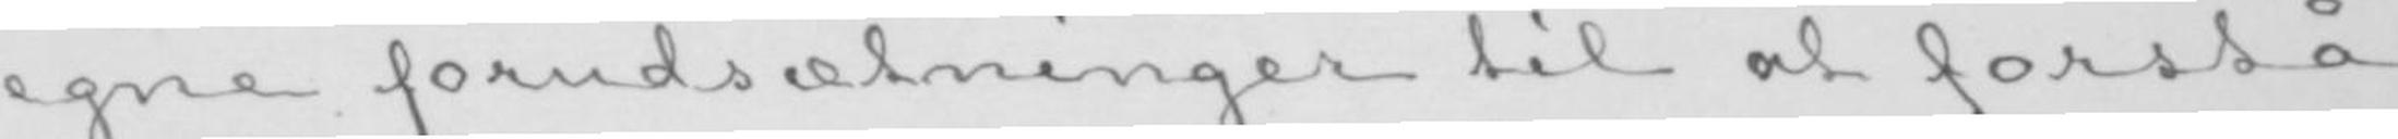egne forudsætninger til at forstå
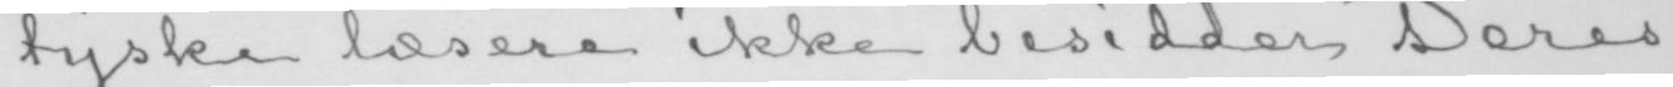tyske læsere ikke besidder Deres
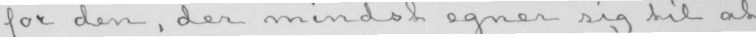for den, der mindst egner sig til at
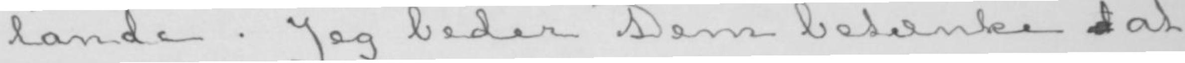lande. Jeg beder Dem betænke d at
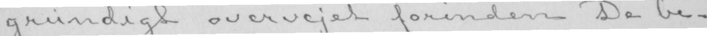grundigt overvejet forinden De be-
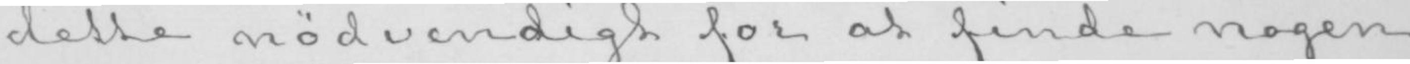dette nødvendigt for at finde nogen
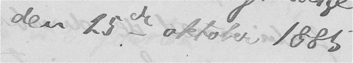den 25de oktober 1885.
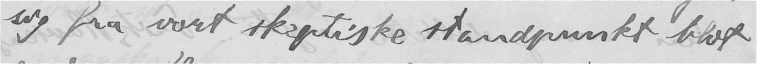sig fra vort skeptiske standpunkt blot
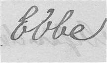Ebbe.
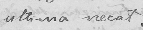ultima necat.
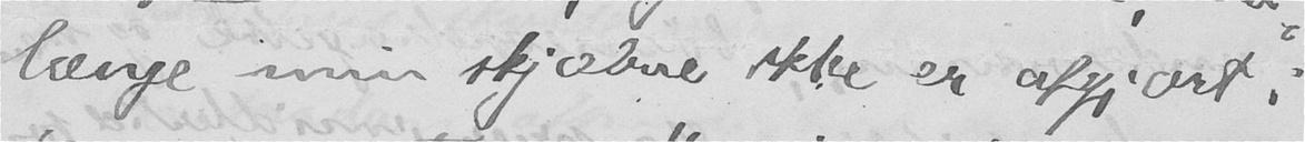længe min skjæbne ikke er afgjort i
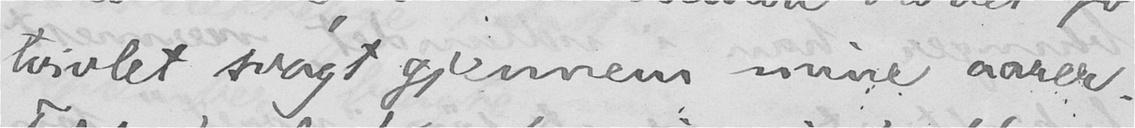tvivlet svagt gjennem mine aarer.
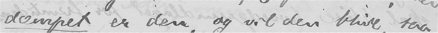dempet er den, og vil den blive, saa-
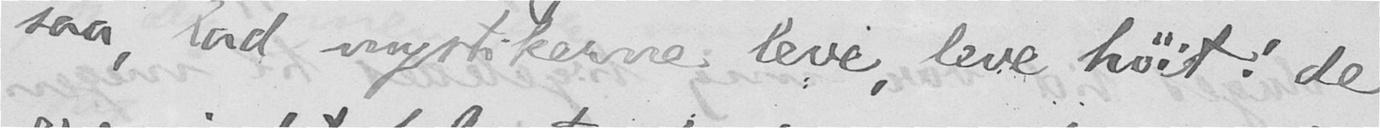saa, lad mystikerne leve, leve høit! de
